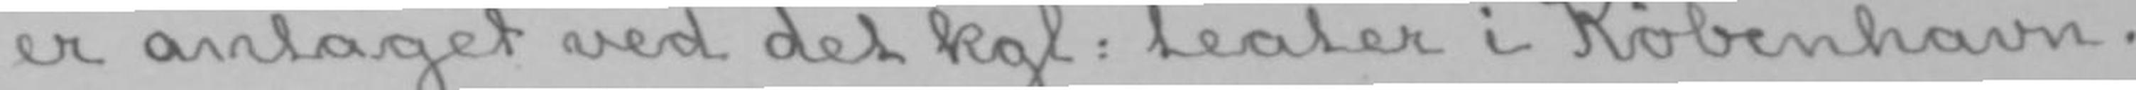er antaget ved det kgl: teater i København.
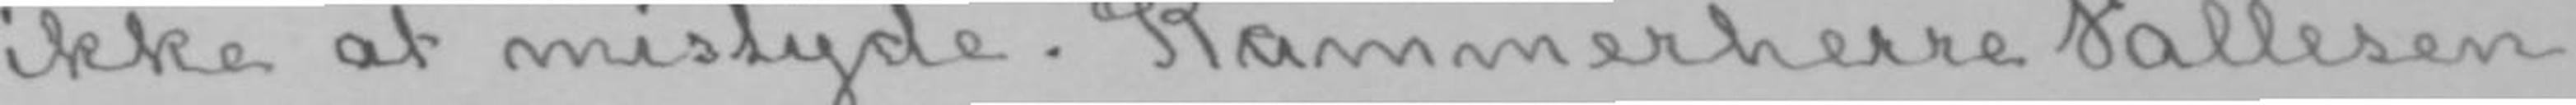ikke at mistyde. Kammerherre Fallesen
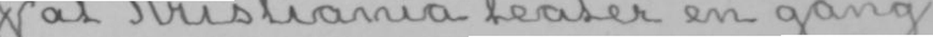at Kristiania teater en gang
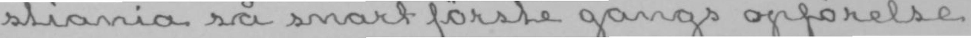stiania så snart første gangs opførelse
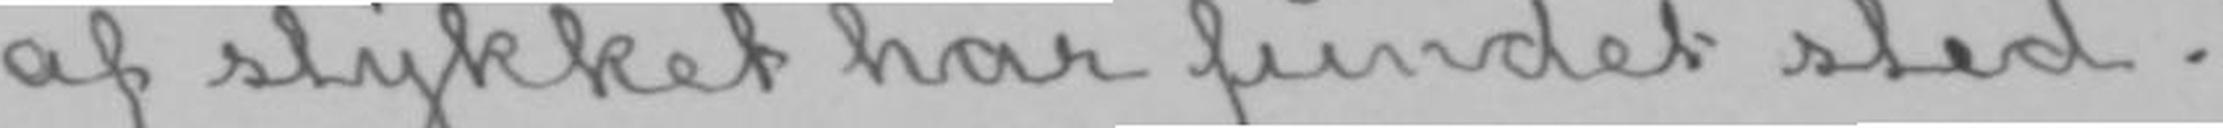af stykket har fundet sted.
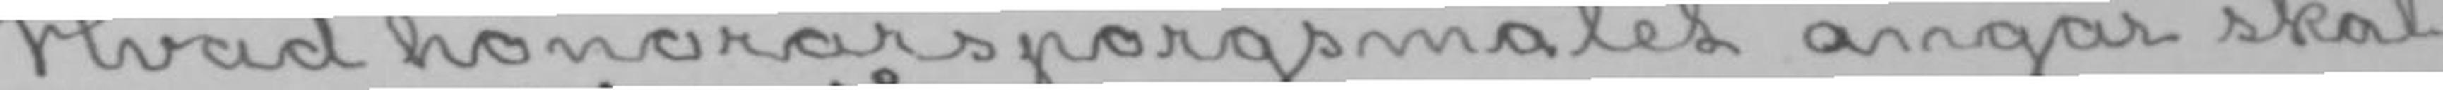Hvad honorarspørgsmålet angår skal
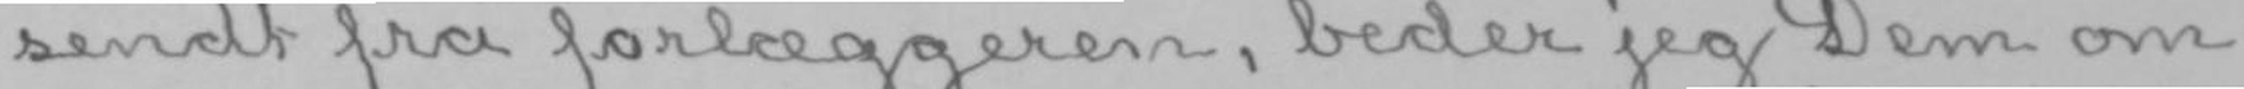sendt fra forlæggeren, beder jeg Dem om
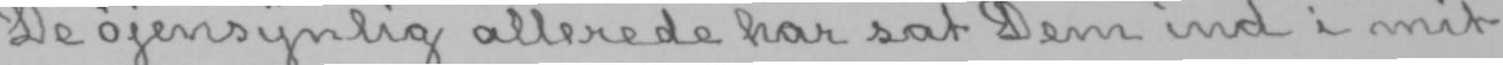De øjensynlig allerede har sat Dem ind i mit
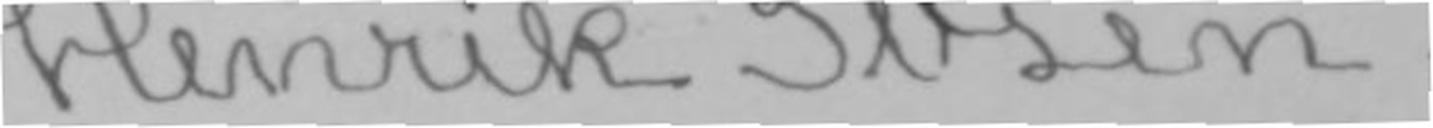Henrik Ibsen.
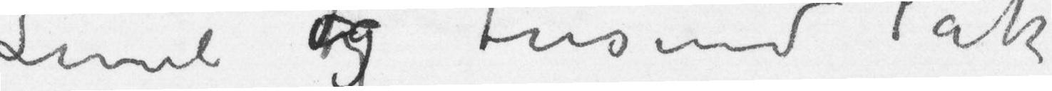Levvel og tusind Tak
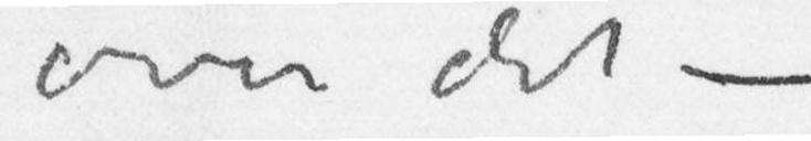over det –
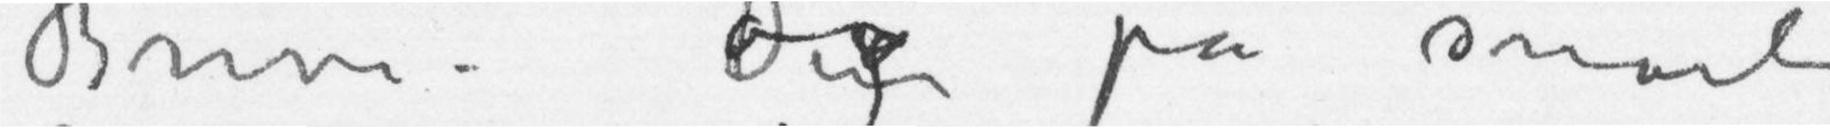Breve. Dinog på snarli
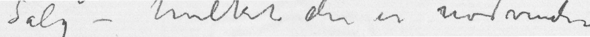Salg – hvilket der er nødvendi
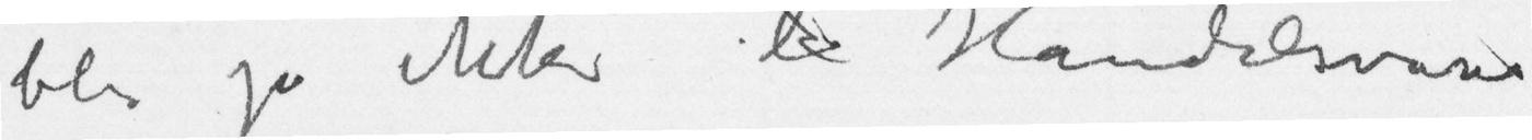blir jo ikkeHandelsvare
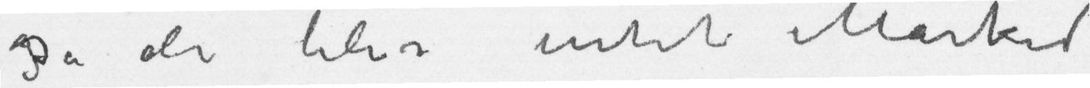også der blir intet Marked
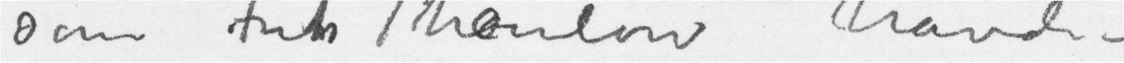som Fritz Thaulow havde –
